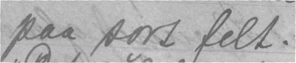paa sort felt.
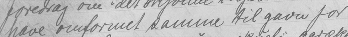have omformet samme til gavn for
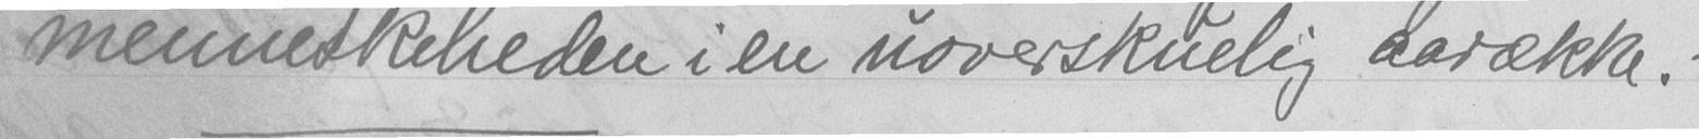menneskeheden i en uoverskuelig aarække. -
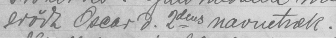erødt Oscar. D. 2dens navnetræk.
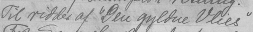Til ridder af "Den gyldne Vlies"
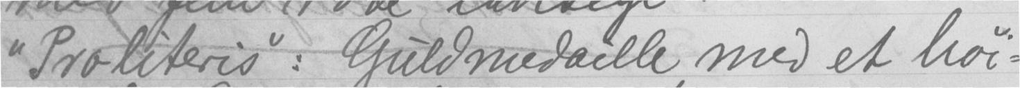"Proliteris": Guldmedaille med et høi-
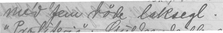med fem røde laksegl.
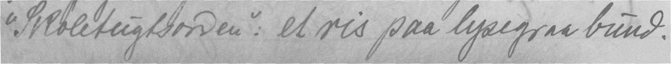"Skoletugtsorden": et ris paa lysegraa bund.
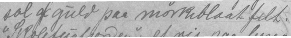sol af guld paa mørkeblaat felt.
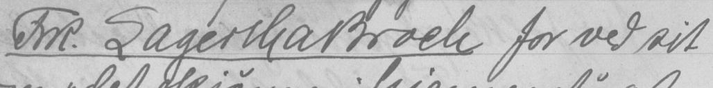Frk. Lagertha Broch for ved sit
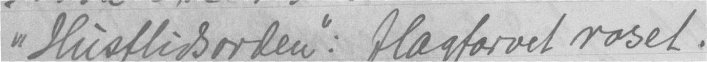"Husflidsorden": flagfarvet roset.
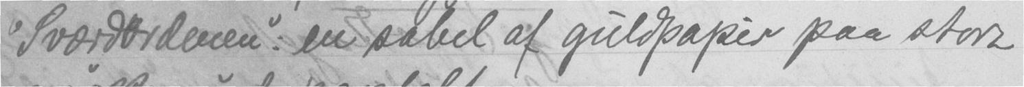"Sværdordenen": en sabel af guldpapir paa stort
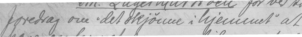foredrag om "det skjønne i hjemmet" at
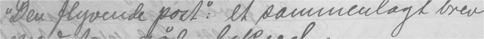"Den flyvende post": et sammenlagt brev
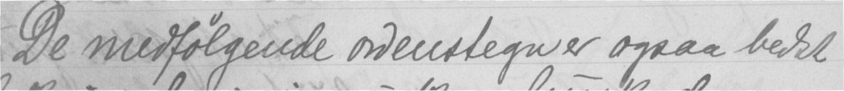De medfølgende ordenstegn er ogsaa bedst
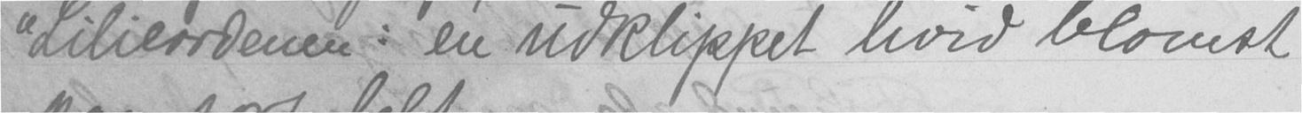"Lilieordenen: en udklippet hvid blomst
